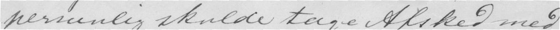personlig skulde tage Afsked med
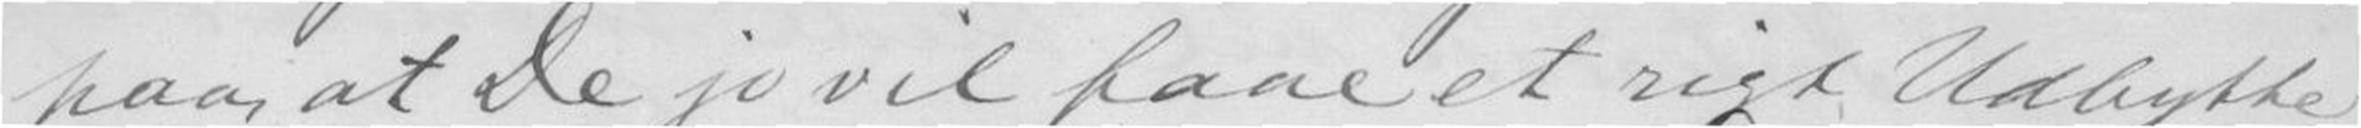paa at De jo vil faae et rigt Udbytte
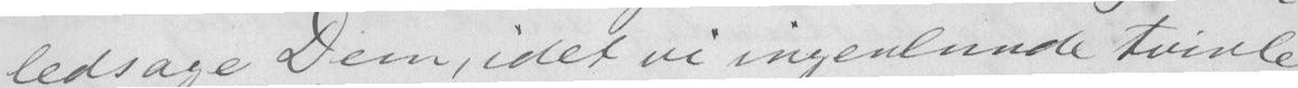ledsage Dem, idet vi ingenlunde tvivle
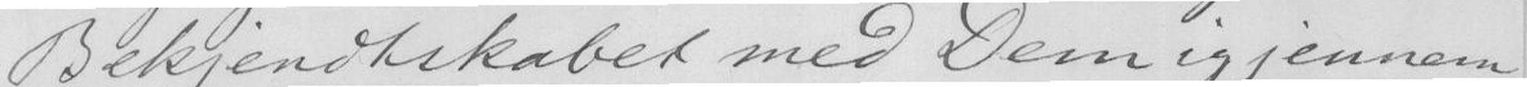Bekjendtskabet med Dem igjennem
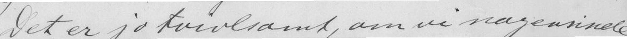Det er jo tvivlsomt, om vi nogensinde
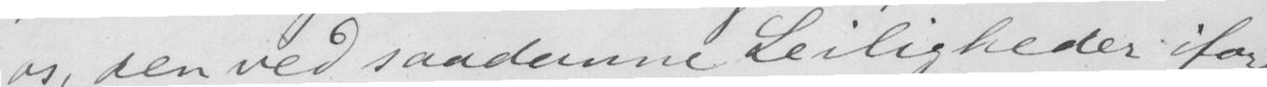os, den ved saadanne Leiligheder ifor
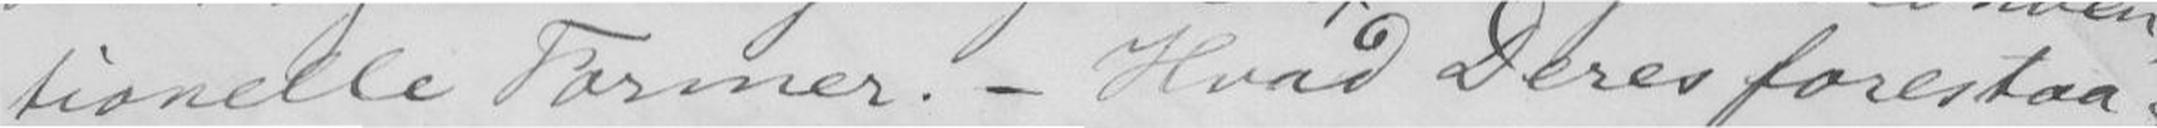tionelle Forner. - Hvad Deres forestaa
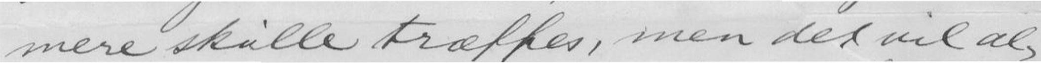mere skulde træffes, men det vil al
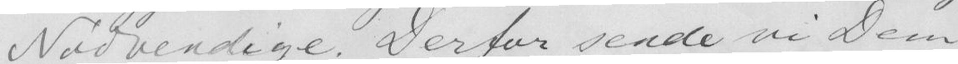Nødvendige, Derfor sende vi Dem
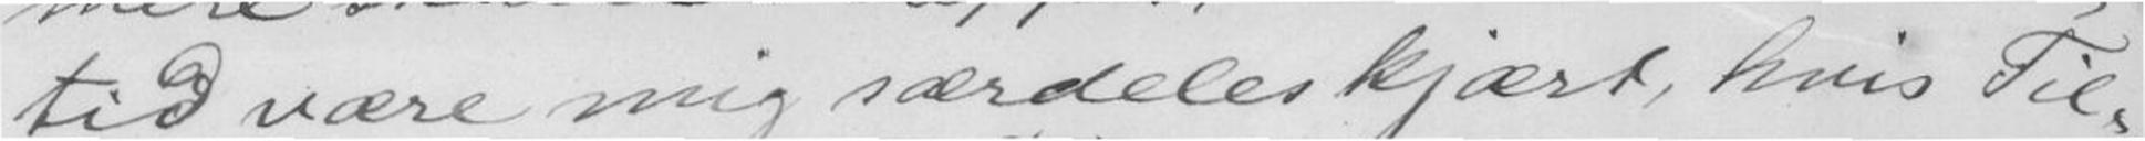tid være mig særdeles kjært, hvis Til
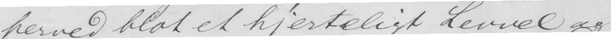herved blot et hjerteligt Levvel og
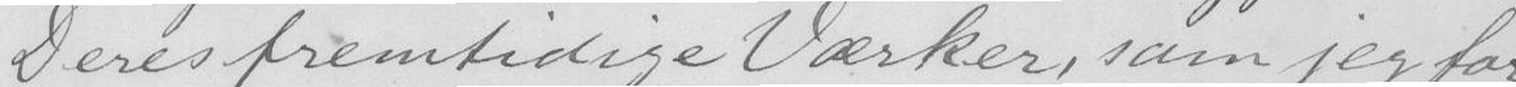Deres fremtidige Værker, som jeg for
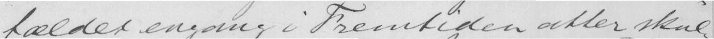fældet engang i Fremtiden atter skul
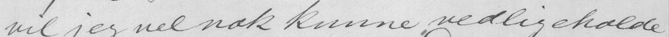vil jeg vel nok kunne vedligeholde
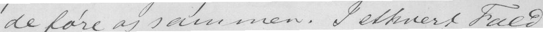de føre os sammen. I ethvert Fald
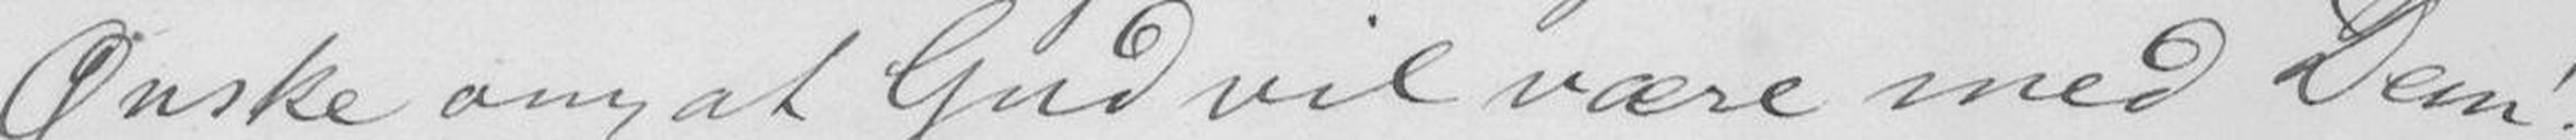Ønske om, at Gud vil være med Dem!
